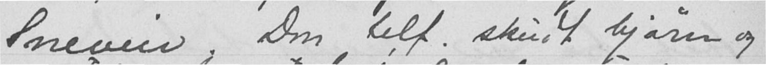Sneveir. Don telf. skudt bjørn og
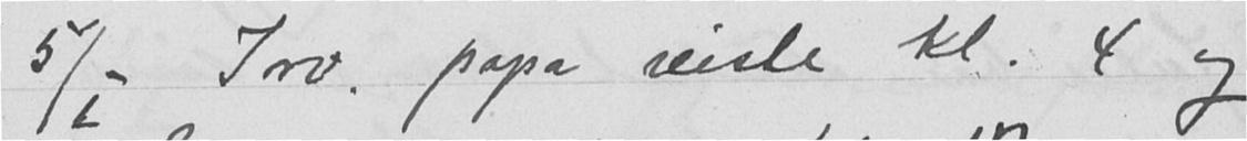5/I Int. papa reiste kl. 4 og
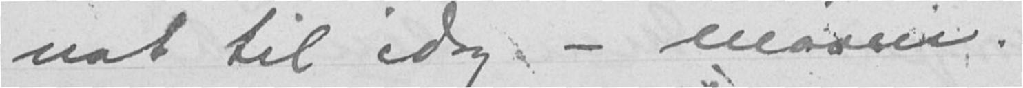nat til idag - maven.
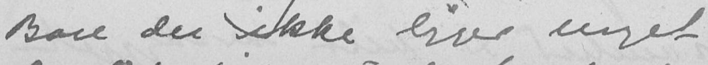Bare der ikke ligger noget
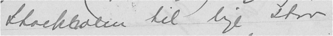Stockholm til lige stor
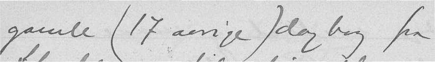gamle (17 aarige) dagbog fra
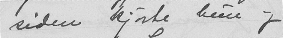siden kjørte hun og
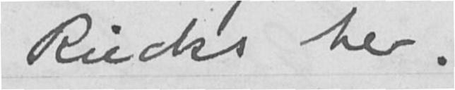Riecks her.
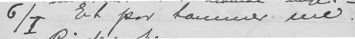6/I Et par tommer sne.
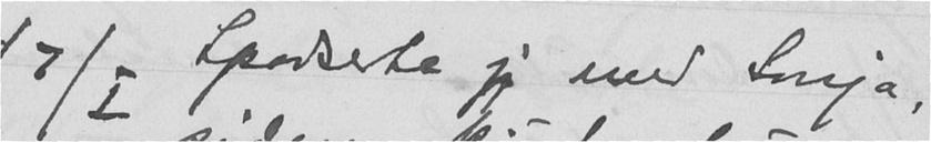7/I Spadserte jeg med Sonja,
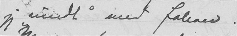jeg rundt med Johan.
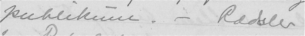publikum. - Rædsler
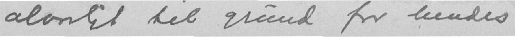alvorligt til grund for hendes
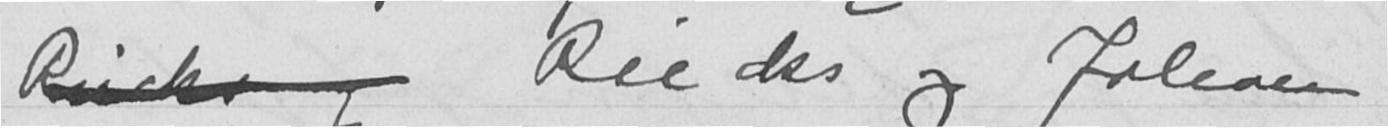Riecks og Riecks og Johan
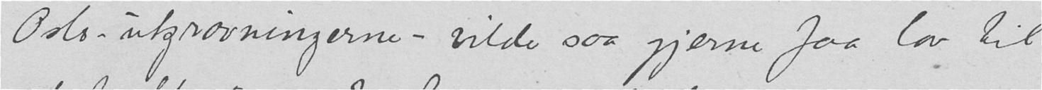Oslo-utgravningerne – vilde saa gjerne faa lov til
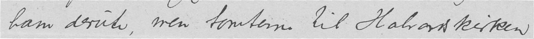ham derute, men tomterne til Halvardskirken,
